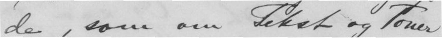de, som om Tekst og Toner
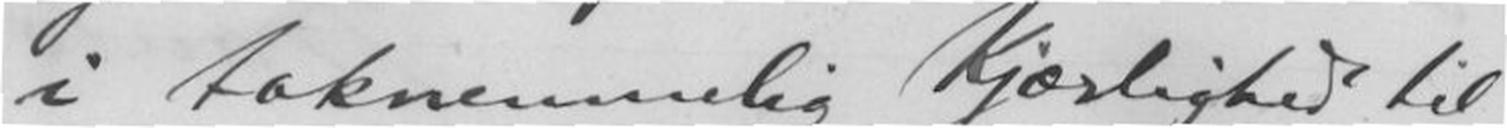i taknemmelig Kjærlighed til
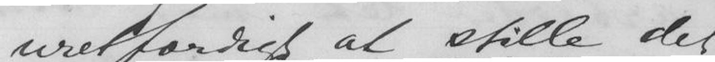uretfærdigt at stille det
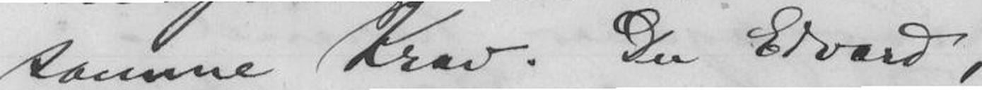samme Krav. Du Edvard,
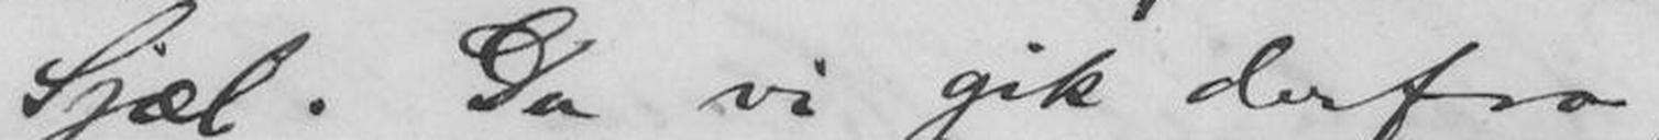Sjæl. Da vi gik derfra,
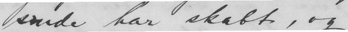sinde har skabt, og
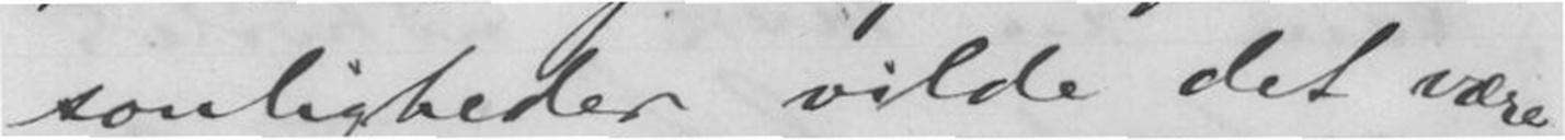sonligheder vilde det være
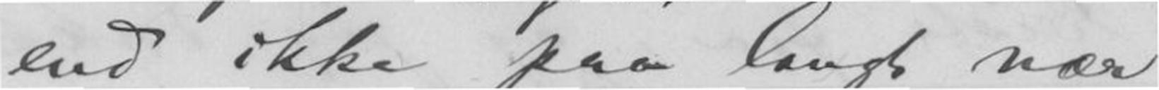end ikke paa langt nær
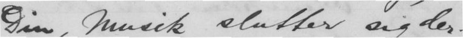Din Musik slutter sig der-
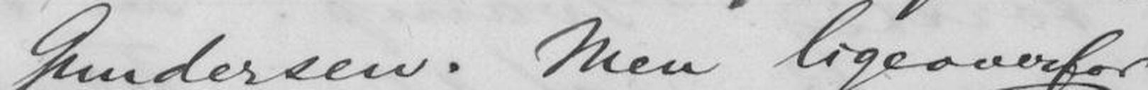Gundersen. Men ligeoverfor
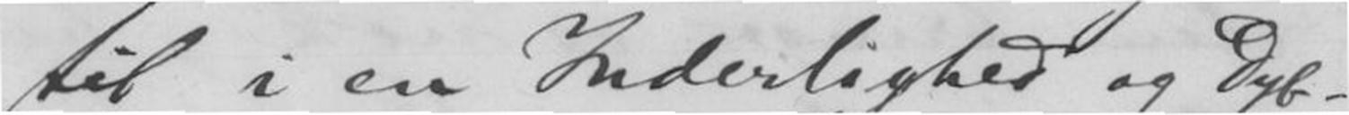til i en Inderlighed og Dyb-
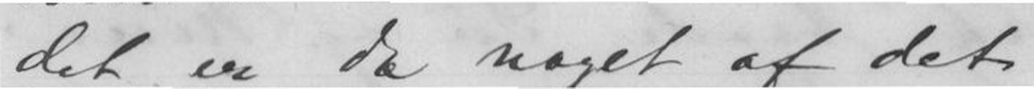det er da noget af det
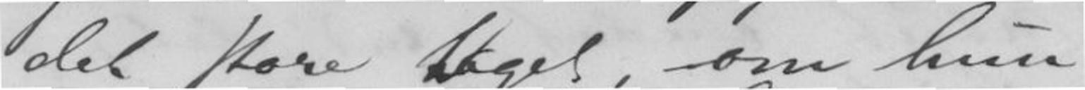det store taget, om hun
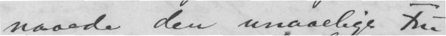naaede den unaaelige Fru
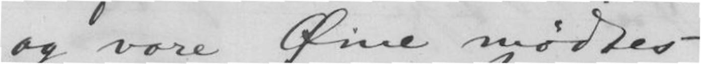og vore Øine mødtes
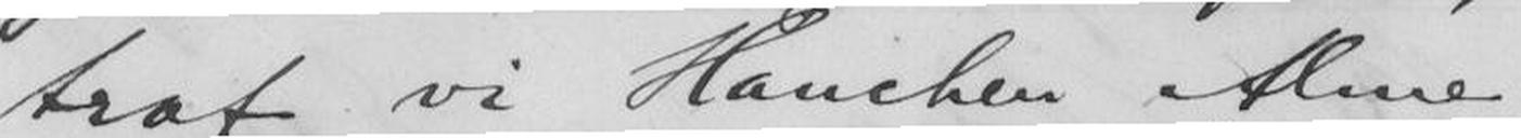traf vi Hanchen Alme,
